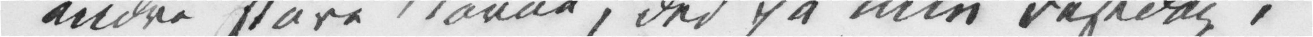andre store Navne, der på min Festdag i
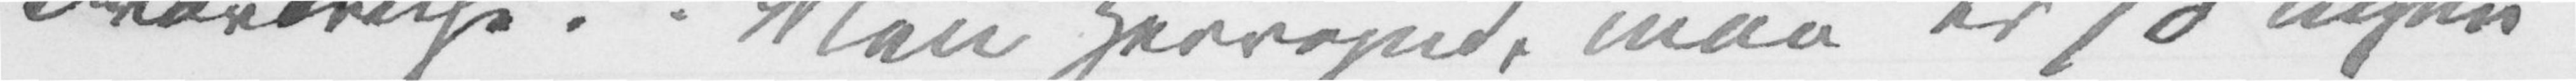Fraværelse. Men Herregud, man er jo ingen
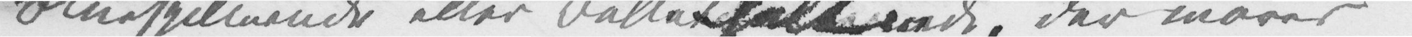Skuespillerinde eller Balletheltinde, der morer
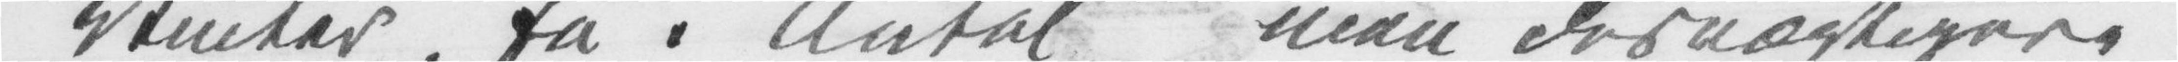Vinter, få i Antal men desvægtigere
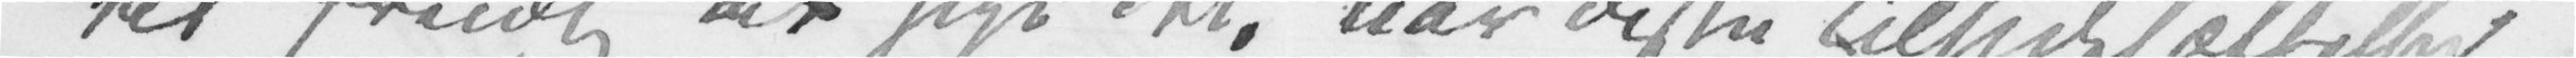til freidig at sige det, når disse Tilsidesættelser
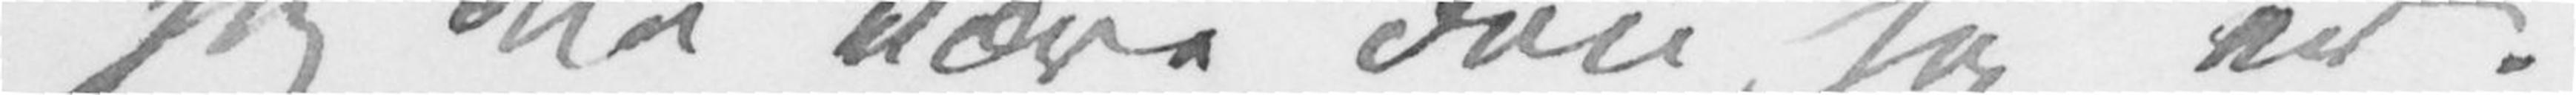jeg ikke være den jeg er.
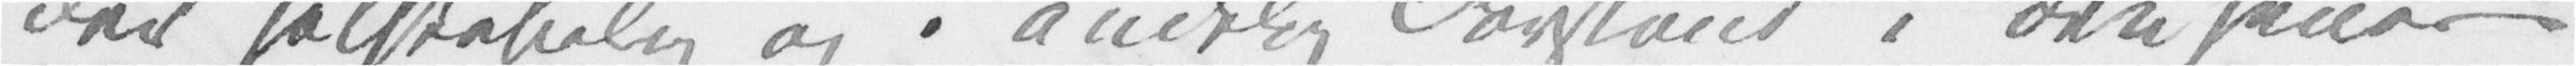der selskabelig og i åndelig Forstand i den senere
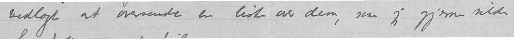vedlagt at oversende en liste over dem, som jeg gjerne vilde
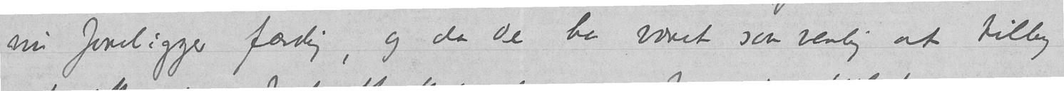nu foreligger færdig, og da de har været saa venlig at tilby
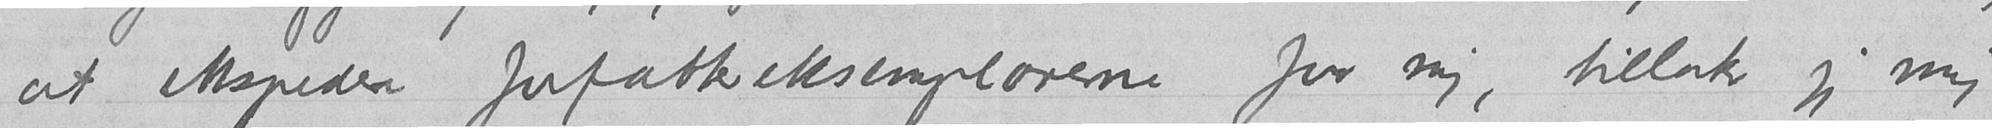at ekspedere forfattereksemplarerne for mig, tillater jeg mig
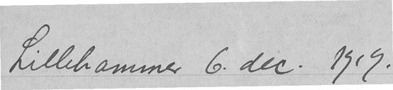Lillehammer 6.dec. 1919.
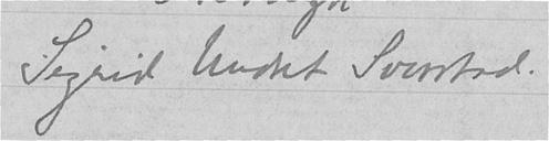Sigrid Undset Svarstad.
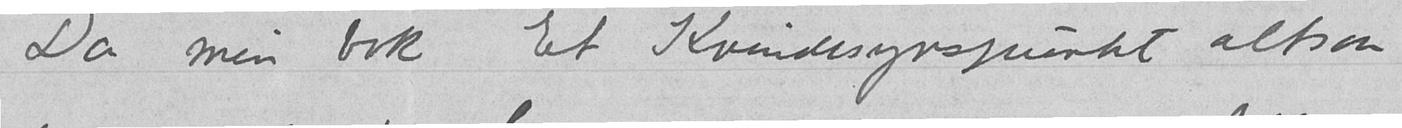Da min bok Et Kvindesynspunkt altsaa
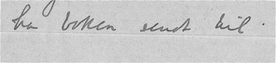ha boken sendt til.
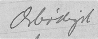Ærbødigst
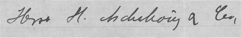Herrer H. Aschehoug & Co,,
